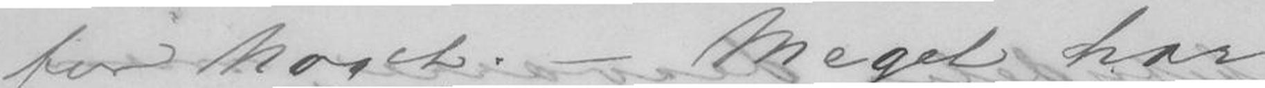for Noget. - Meget har
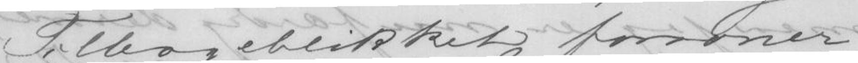Tilbageblikket forsoner
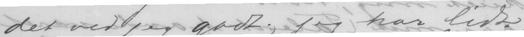det ved jeg godt; jeg har lidt
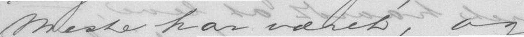Meste har været, og
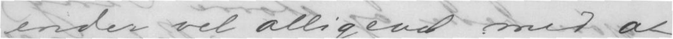ender vel alligevel med at
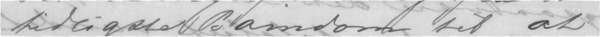tidligste Barndom til at
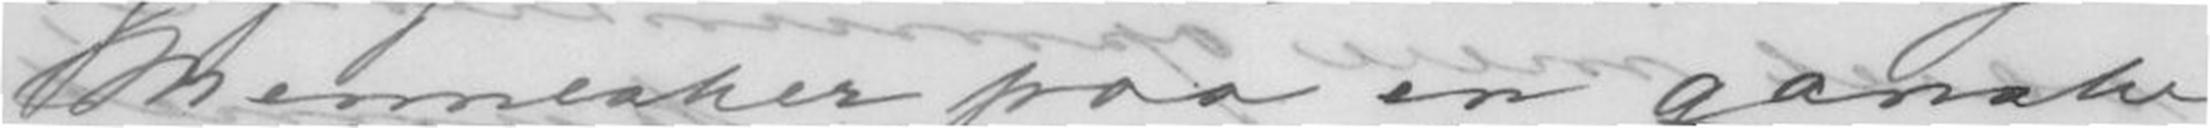Mennesker paa en ganske
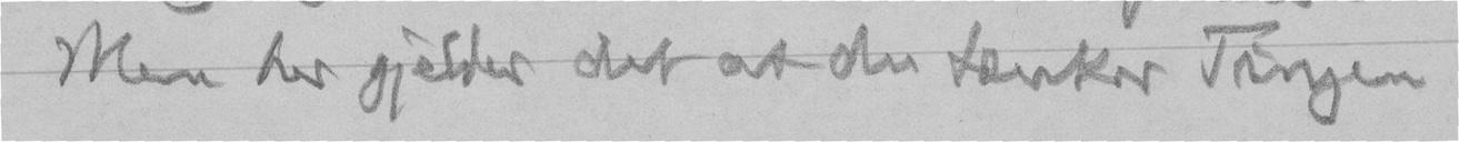Men her gjelder det at du tænker Tingen
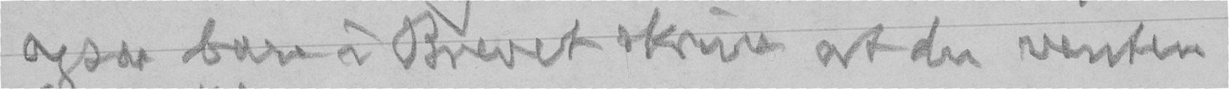ogsaa bare i Brevet skrive at du venter
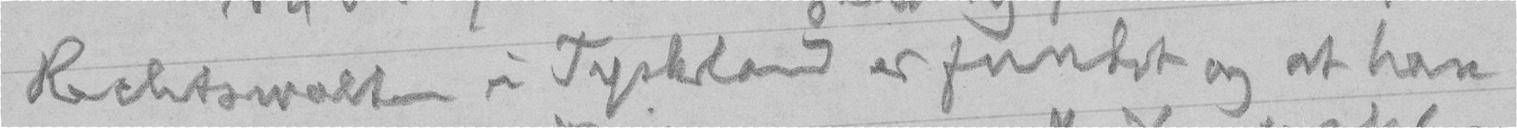Rechtswalten i Tyskland er fundet og at han
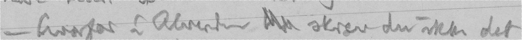- hvorfor i Alverden  skrev du ikke det
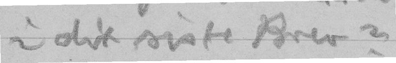i dit siste Brev?
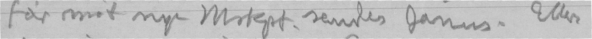før mit nye Mskpt. sendes Janes. Eller
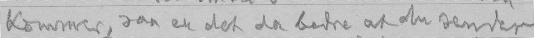kommer, saa er det da bedre at du sender
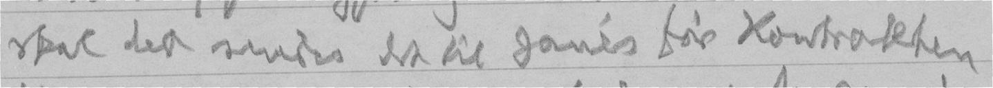skal det sendes det til Janés før Kontrakten
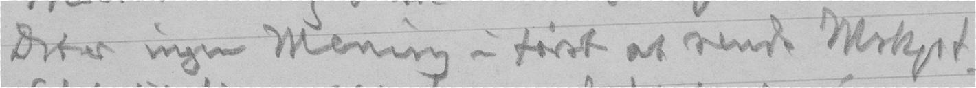Det er ingen Mening i først at sende Mskpt.
